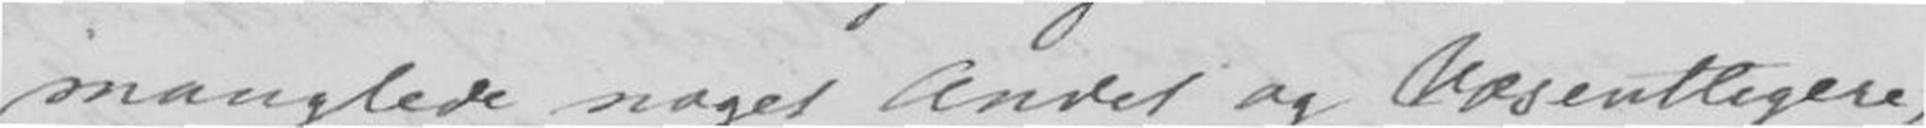manglede noget Andet og Væsentligere,
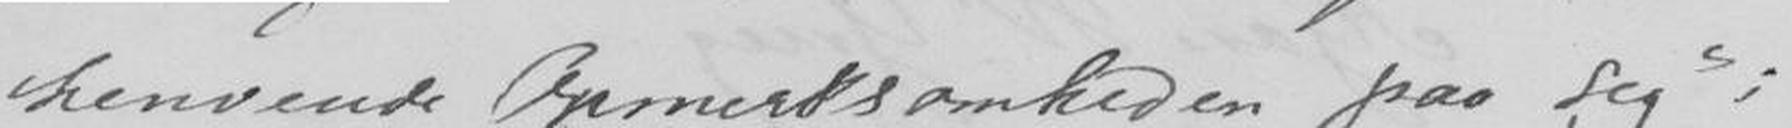henvende Opmerksomheden paa sig;
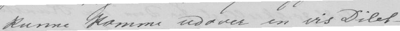kunne komme udover en vis Dilet-
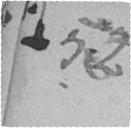52
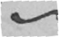-
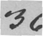§ 36.
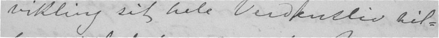vikling sit hele Verdkusliv til-
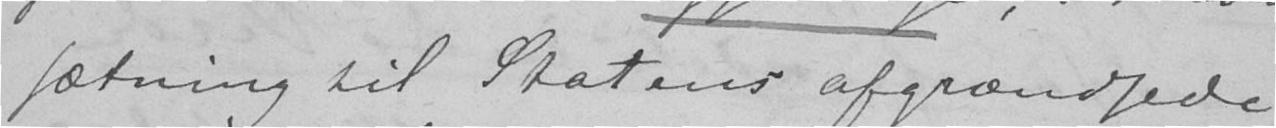sætning til Statens afgrændsede
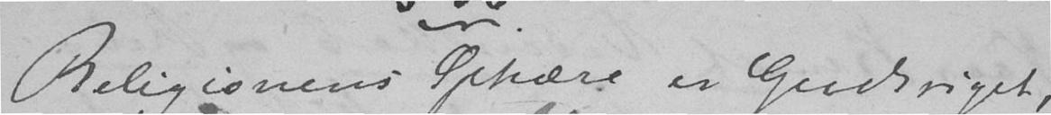Religionens Sphære er Gudsriget,
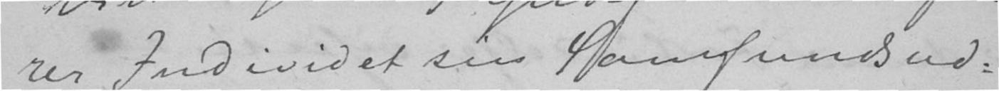rer Individet sin Samfundsud-
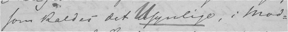som kaldes det synlige, i Mod-
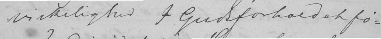Virkelighed. I Gudsforholdet fø-
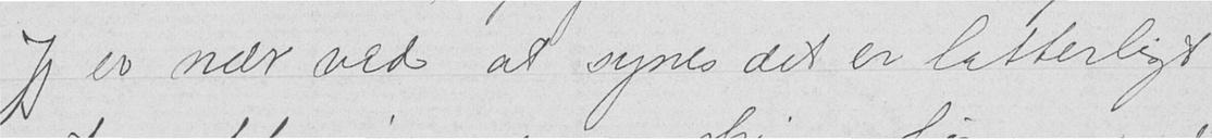Jeg er nær ved at synes det er latterligt
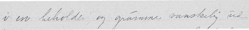i en beholder, og grumme vanskelig ud.
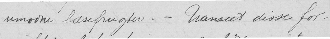umodne læsefrugter. - Uanseet disse for-
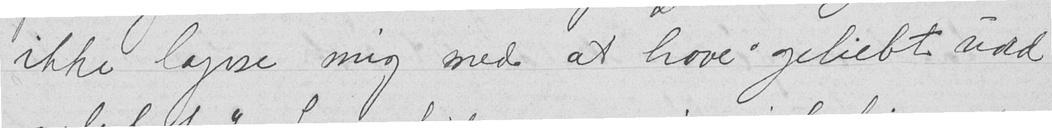ikke lapse mig med at have "geliebt und
